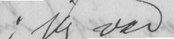jeg var
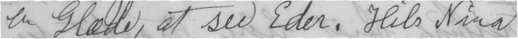en Glæde, at see Eder. Hils Nina
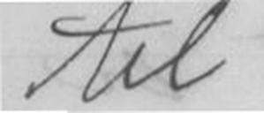til
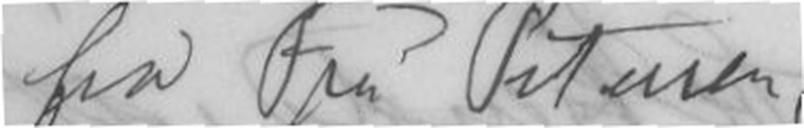fra Fru Petersen,
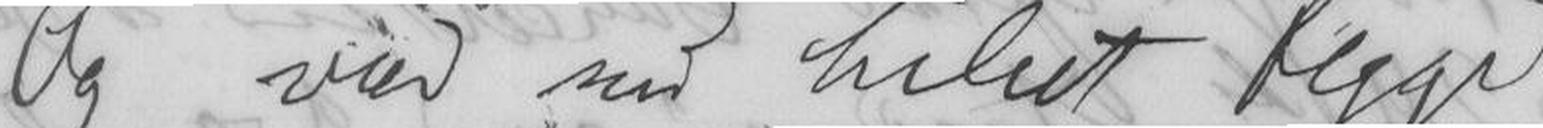Og vær nu hilset Begge
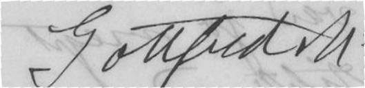Gottfred
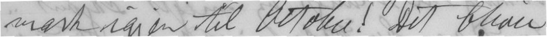mark igjen til October ! Det blive
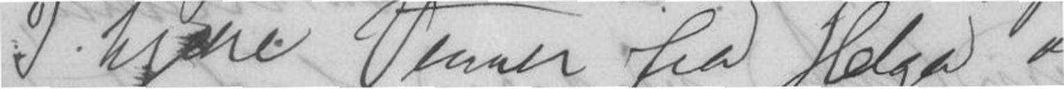I kjære Venner fra Helga og
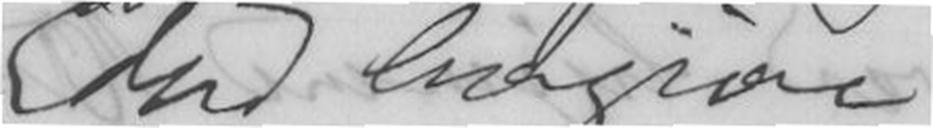Eder hengivne
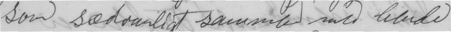som sædvanligt sammen med hende
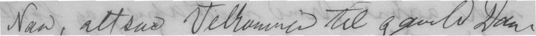Naa, altsaa Velkommen til gamle Dan-
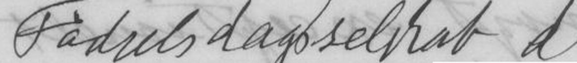Fødselsdagsselskab d
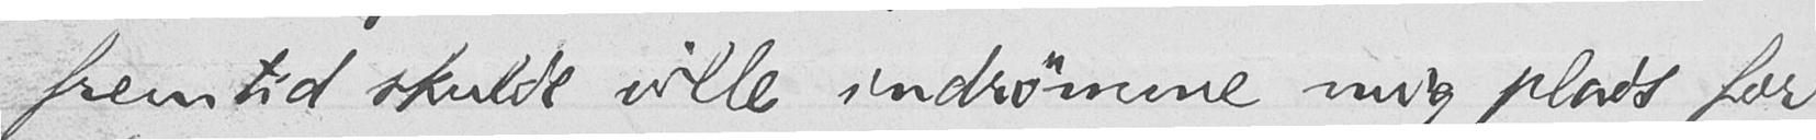fremtid skulde ville indrømme mig plads for
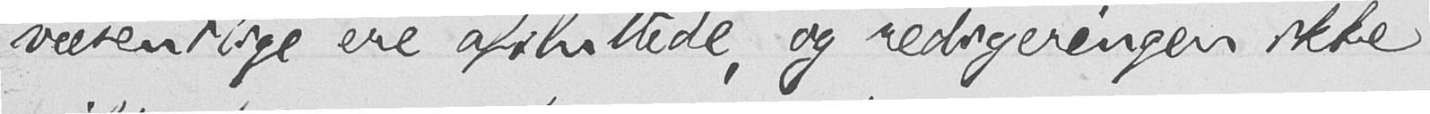væsentlige ere afsluttede, og redigeringen ikke
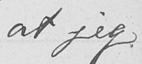at jeg
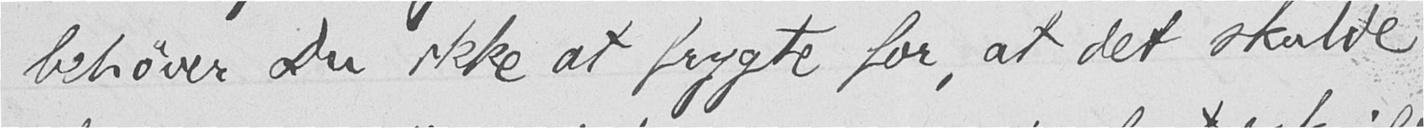behøver Du ikke at frygte for, at det skulde
